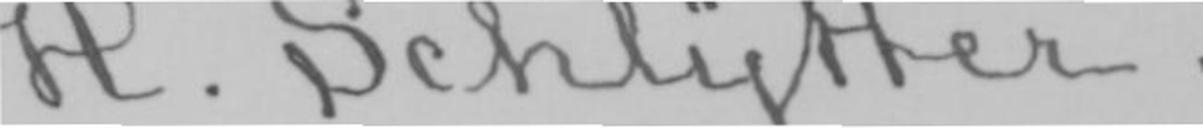H. Schlytter.
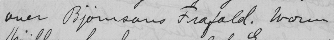over Bjørnsons Frafald. Worm
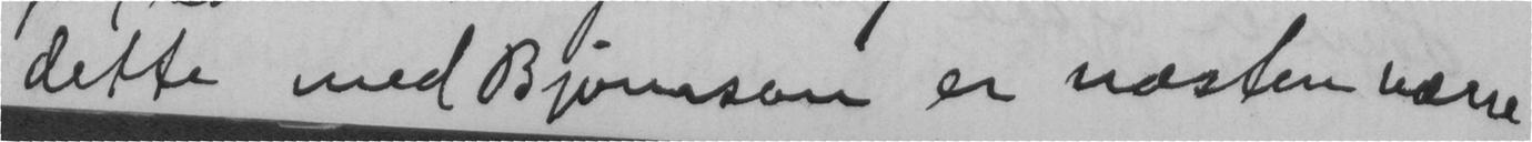dette med Bjørnson er næsten værre
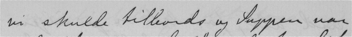vi skulde tilbords og Suppen var
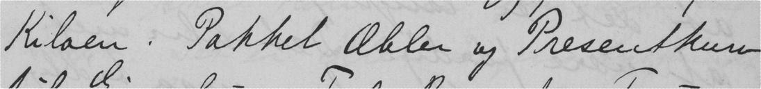Kiloen. Pakket Æbler og Presentkurv
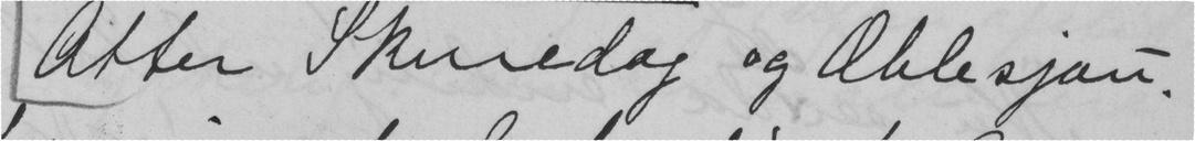Atter Skuredag og Æblesjau.
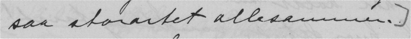saa storartet allesammen.
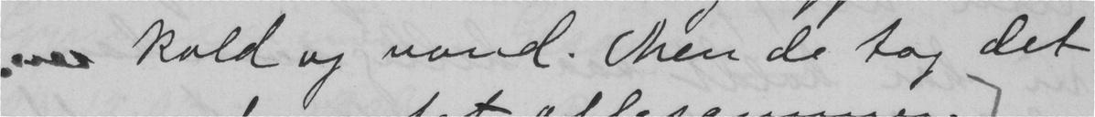kald og vond. Men de tog det
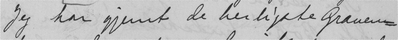Jeg har gjemt de heiligste Graven-
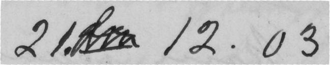21. 12.03
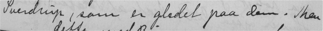Sverdrup, som er gledet paa dem. Men
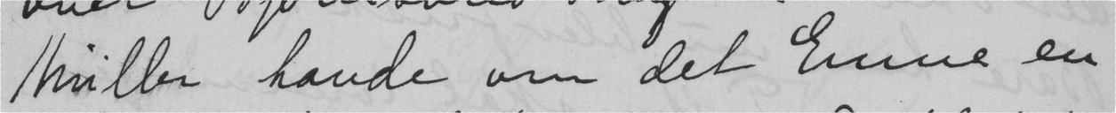Müller havde om det Emne en
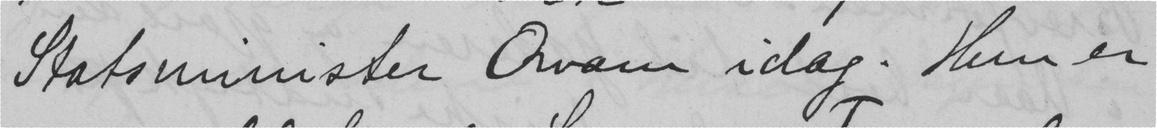Statsminister Qvam idag. Hun er
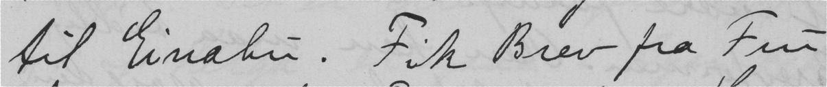til Einabu. Fik Brev fra Fru
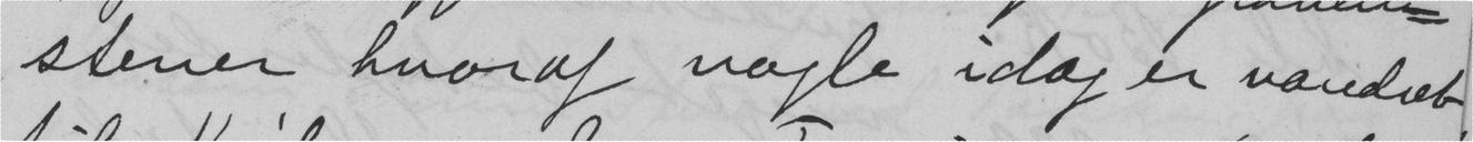stener hvoraf nogle idag er vandret
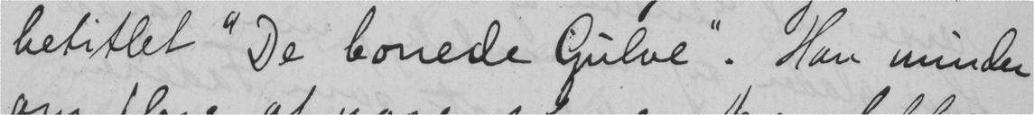betitlet "De bonede Gulve". Han minder
